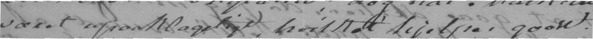været upaaklageligt, hvilket hjelper godt.
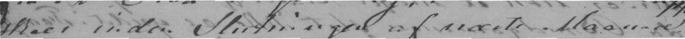skeer inden Slutningen af næste Maaned.
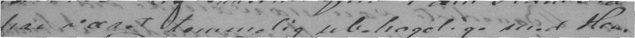har været temmelig ubehagelige med Hen-
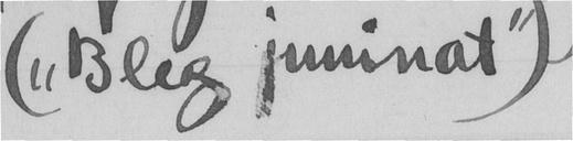("Bleg juninat")
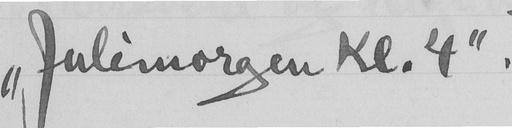"Julimorgen Kl.4".
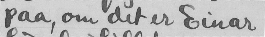paa, om det er Einar
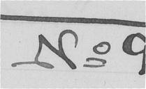No 9
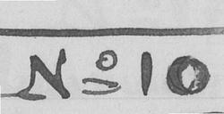No 10
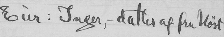Eier: Inger, - datter af fru Høst
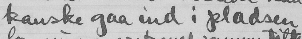kanske gaa ind i pladsen
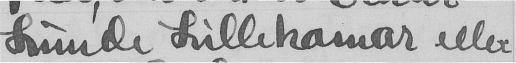Lunde Liillehamar eller
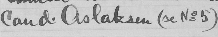Cand. Aslaksen (se No 5)
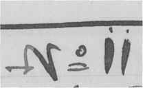No 11
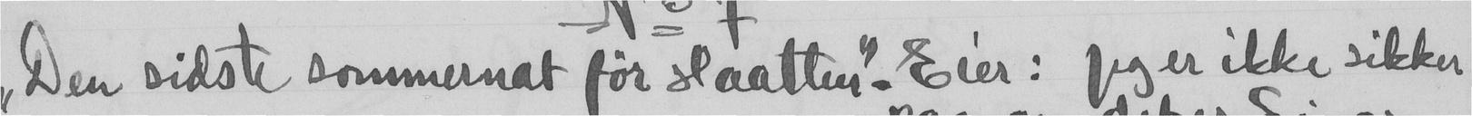"Den sidste sommernat for slaatten". Eier: Jeg er ikke sikker
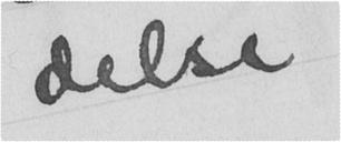delse
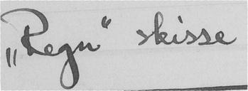"Regn" skisse
